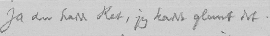Ja du hadde Ret, jeg hadde glemt det.
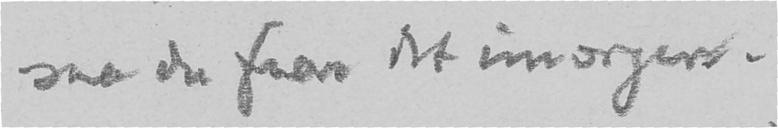saa du faar det imorgen.
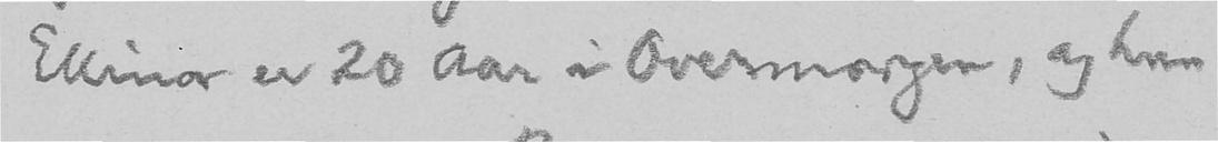Ellinor er 20 Aar i Overmorgen, og hun
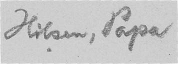Hilsen, Papa
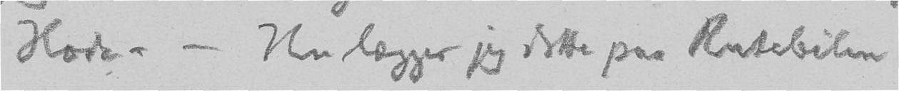Hode. - Nu lægger jeg dette paa Rutebilen
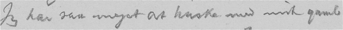Jeg har saa meget at huske med mit gamle
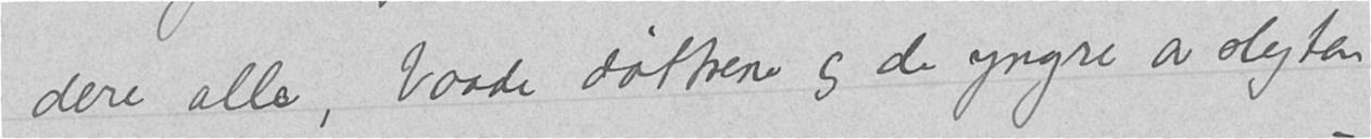dere alle, baade døttrene og de yngre av slegten
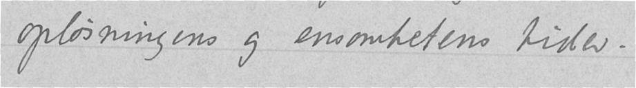opløsningens og ensomhetens tider.
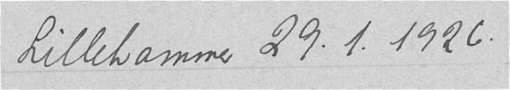Lillehammer 29.1.1926.
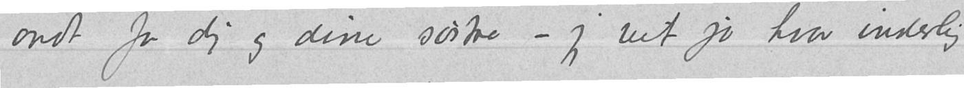ondt for dig og dine søstre – jeg vet jo hvor inderlig
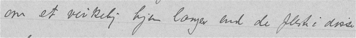om et virkelig hjem længer end de fleste i disse
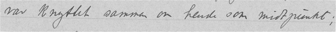var knyttet sammen om hende som midtpunkt;
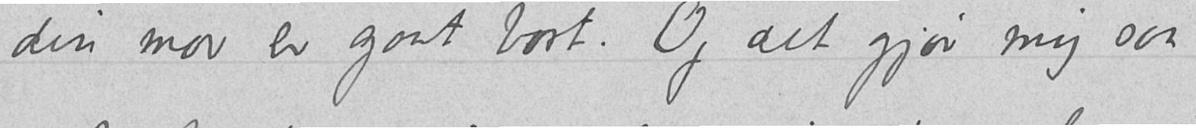din mor er gaat bort. Og det gjør mig saa
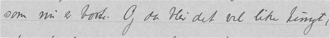som nu er borte. Og da blir det vel like tungt,
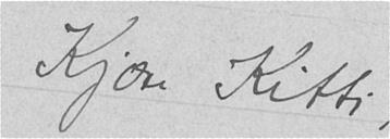Kjære Kitti,
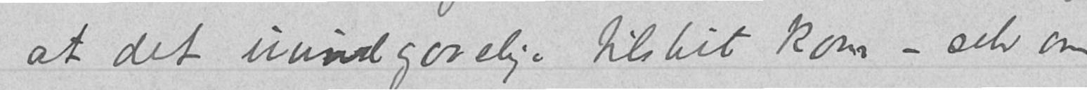at det uundgaaelige tilslut kom - selv om
